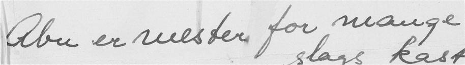Abu er mester for mange
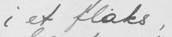i et flaks,
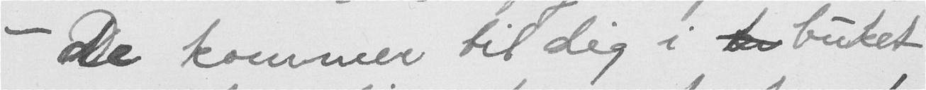De kommer til dig i buket
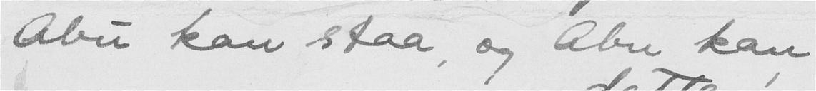Abu kan staa, og Abu kan
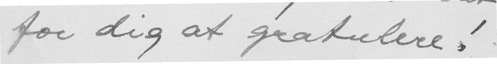for dig at gaatulere!
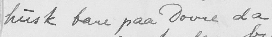husk bare paa Dovre da
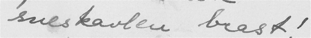snes kavlen brast!
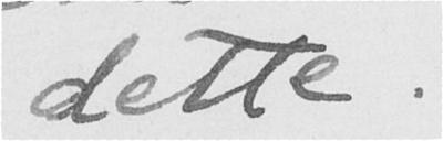dette.
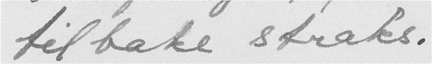tilbake streks.
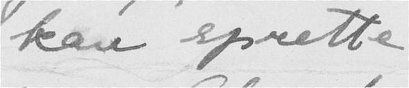kan sprette
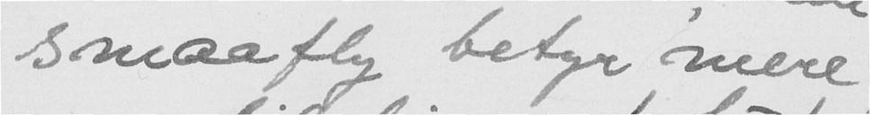smaafly betyr mere
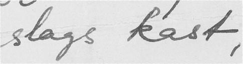slags kast,
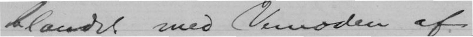blandet med Vemoden af,
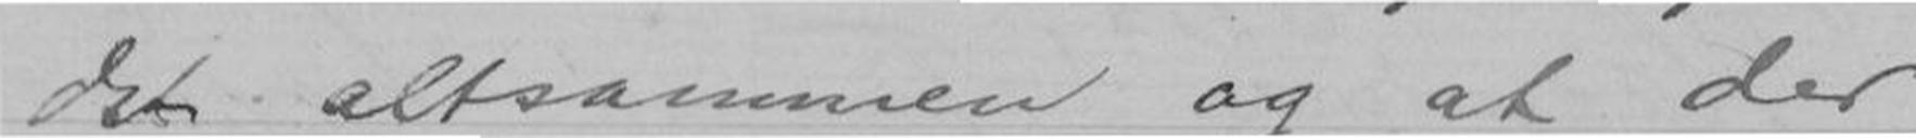det altsammen og at der
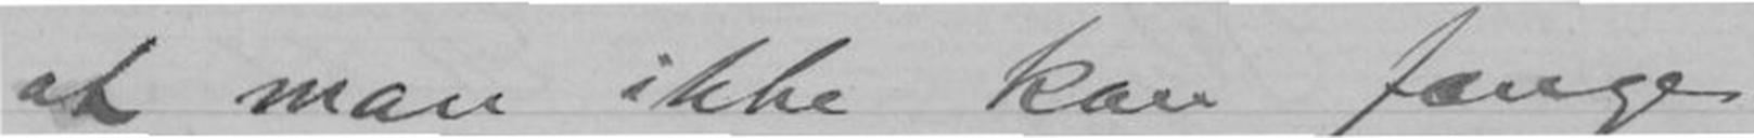at man ikke kan fange
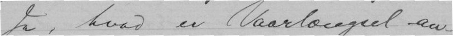Ja, hvad er Vaarlængsel an-
